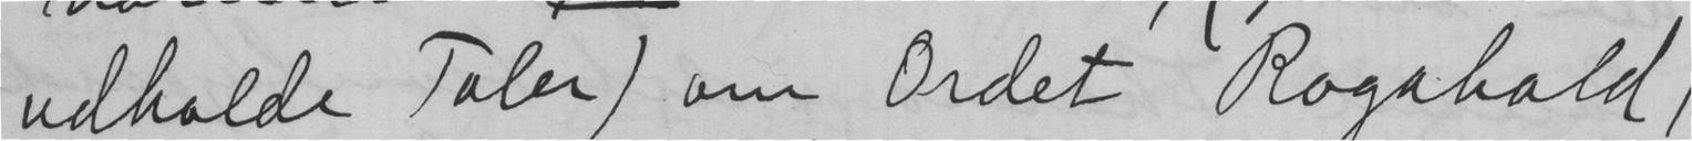udholde Taler) om Ordet Rogabald,
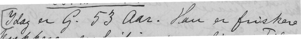Idag er G. 53 aar. Han er friskere
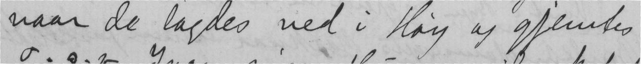naar de lagdes ved i Høy og gjemtes
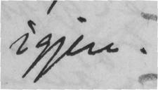igjen.
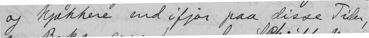og kjækkere end i fjor paa disse Tider,
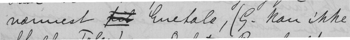nærmest  Enetale, (G. kan ikke
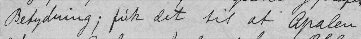Betydning; fik det til at Apalen
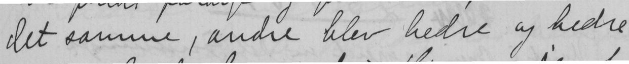det samme, andre blev bedre og bedre
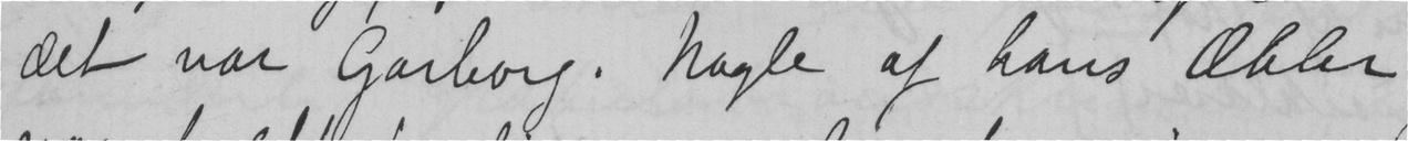det var Garborg. Nogle af hans Æbler
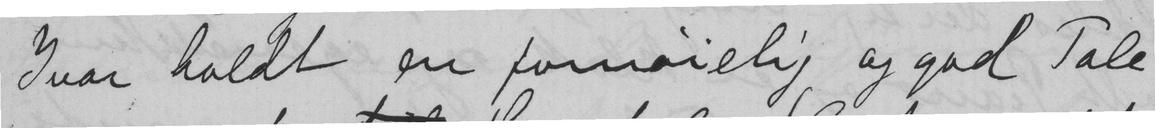Ivar holdt en fornøielig og god Tale
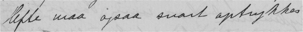Hefte maa ogsaa snart optrykkes
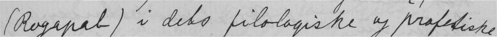(Rogapal) i dets filologiske og profetiske
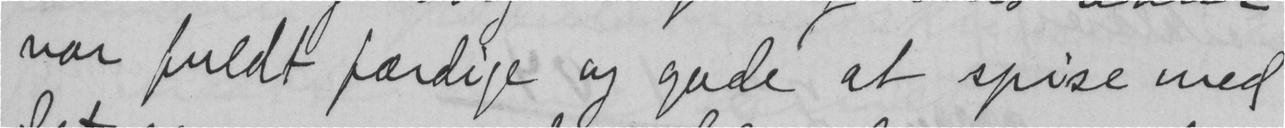var fuldt færdige og gode at spise med
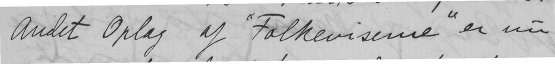Andet Oplag af "Folkeviserne" er nu
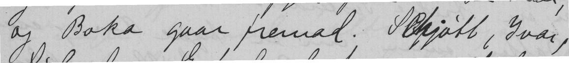og Boka gaar fremad. Schjøtt, Ivar,
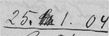25. 1.04
